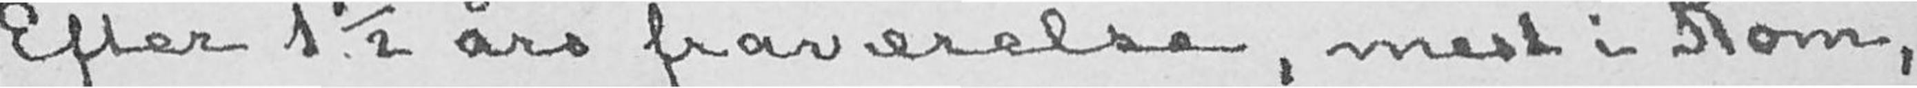Efter 1½ års fraværelse, mest i Rom,
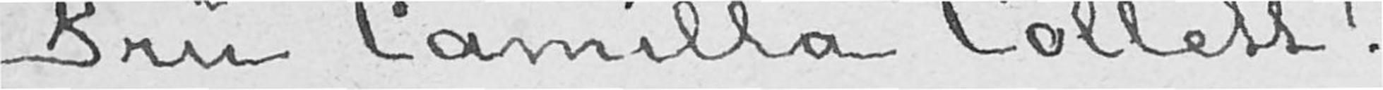Fru Camilla Collett!
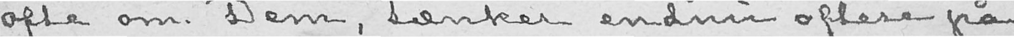ofte om Dem, tænker endnu oftere på
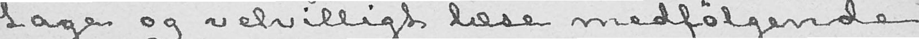tage og velvilligt læse medfølgende
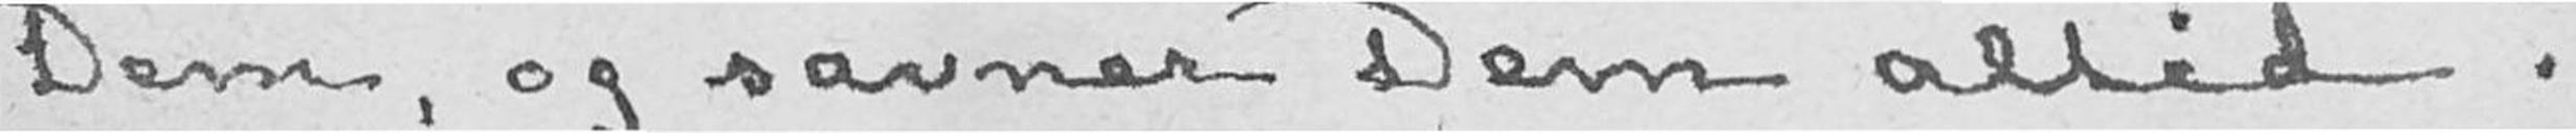Dem, og savner Dem altid.
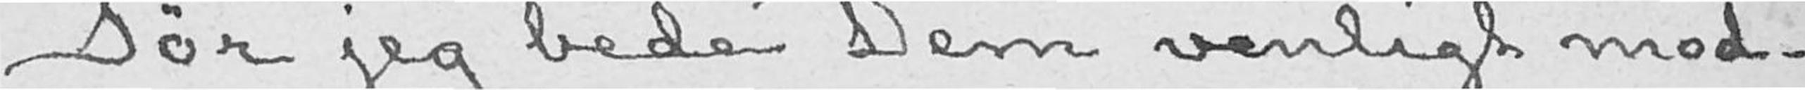Tør jeg bede Dem venligt mod-
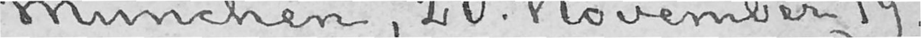München, 20. November 79.
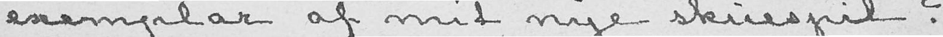exemplar af mit nye skuespil?
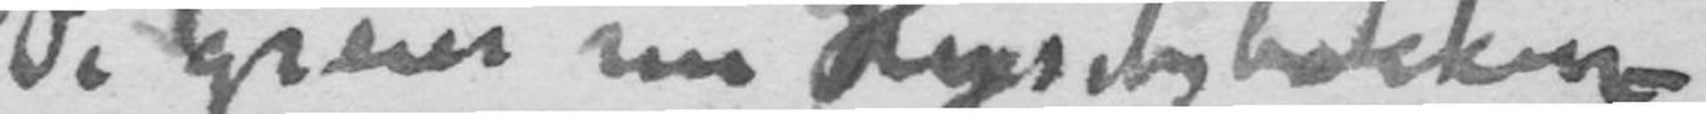Vi greier nu Husebybakken –
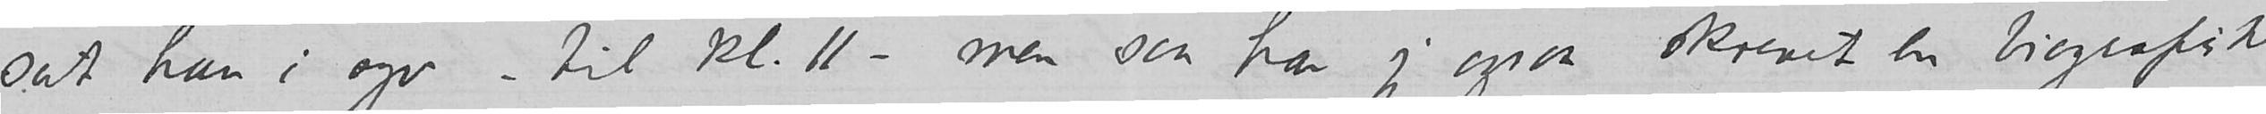sat han i syv – til kl. 11 – men saa har jeg ogsaa skrevet en biografisk
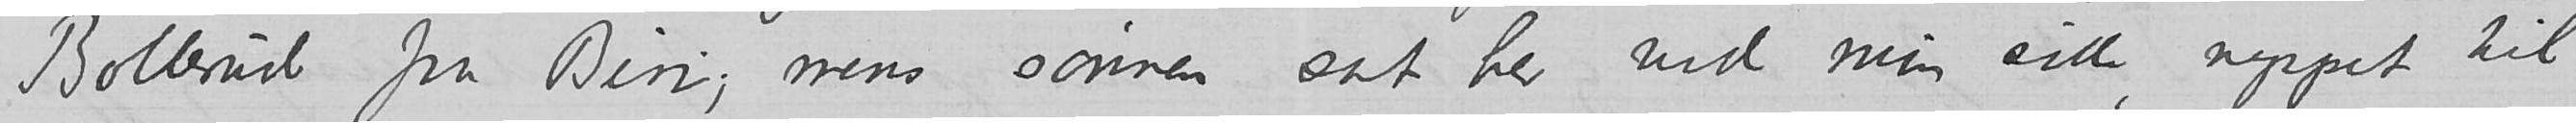Bollerud fra Biri; mens sønnen sat her ved min side, nippet til
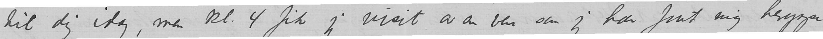til dig i dag, men kl. 4 fik jeg visit av en ven som jeg har faat mig heroppe,
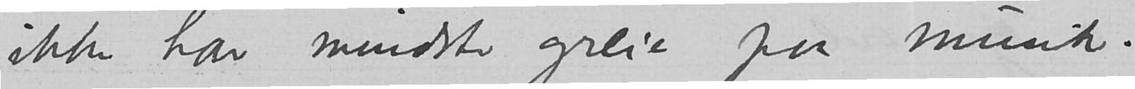ikke har mindste greie paa musik.
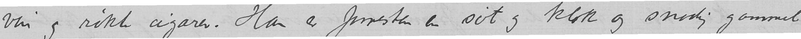vin og røkte cigarer. Han er forresten en søt og klok og snodig gammel
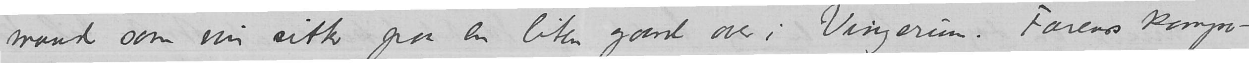mand som nu sitter paa en liten gaard over i Vingerum Farens kompon
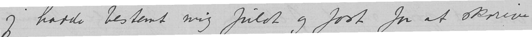jeg hadde bestemt mig fuldt og fast for at skrive
